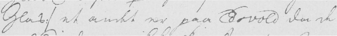Glas :/ et andet er paa Esvold da de
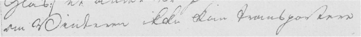om Vinteren ikke kan transportere
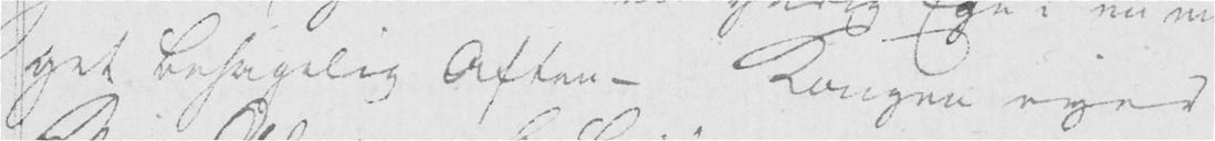get behagelig Aften - Kongen eyed
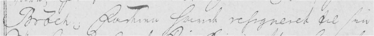Broch; Faderen havde resigneret til sin
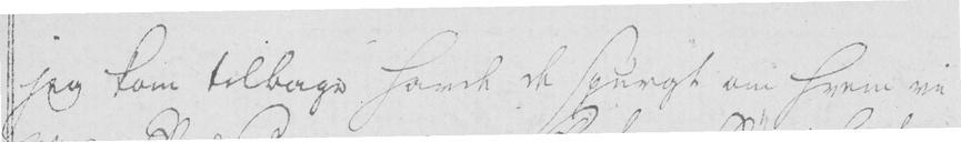jeg kom tilbage havde de spurgt om hvem vi
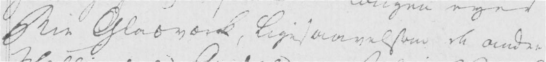Biri Glasværk, ligesaavelsom de andre i
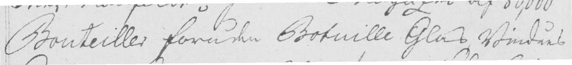Bouteiller foruden Botuille Glas, Vindues
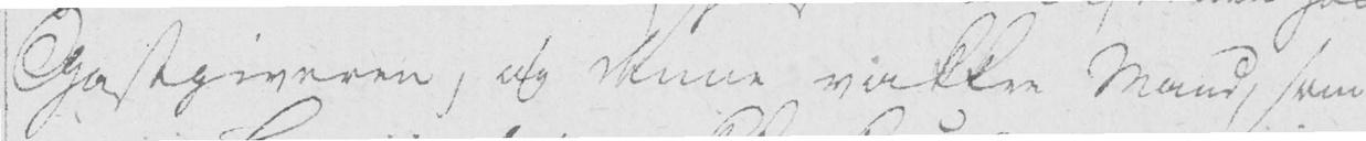Gæstgiveren, og denne vakkre Mand, som
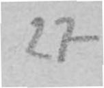27
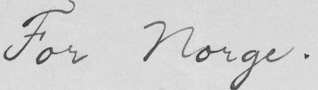For Norge.
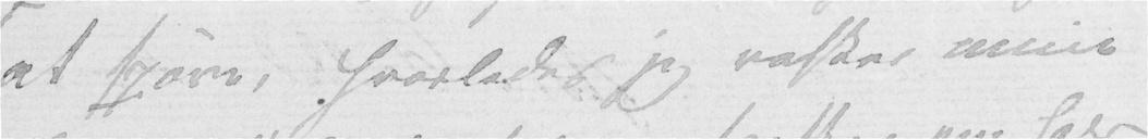at spore, hvorledes jeg vasker mine
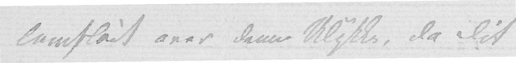lamslået over denne Ulykke, da Dit
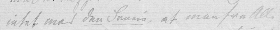intet mod «den Ironie, at man fra Alle
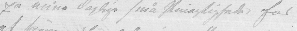på mine daglige små Pinagtigheder for
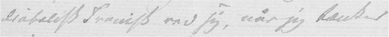diabolisk Ironisk ved sig, når jeg tænker
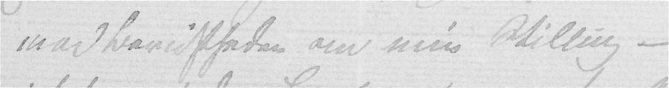mod Bevidstheden om min Stilling –
